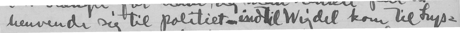henvende sig til politiet - indtil Wigdel kom til Lys-
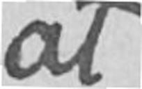at
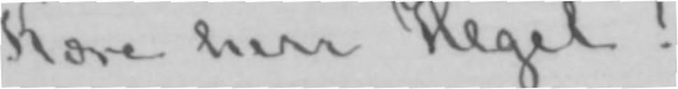Kære herr Hegel!
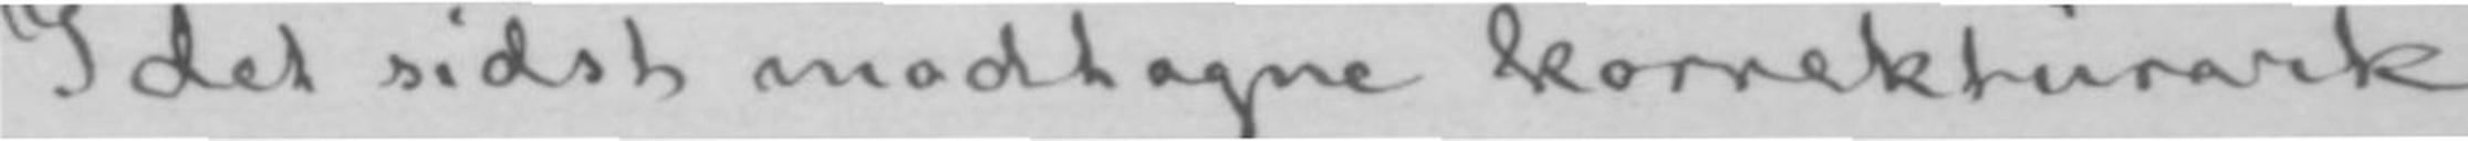I det sidst modtagne korrekturark
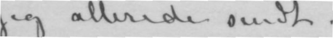jeg allerede sendt.
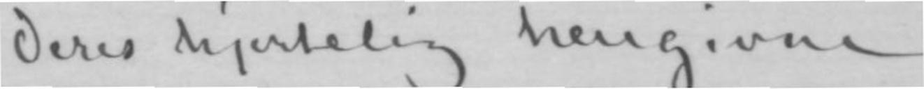Deres hjertelig hengivne
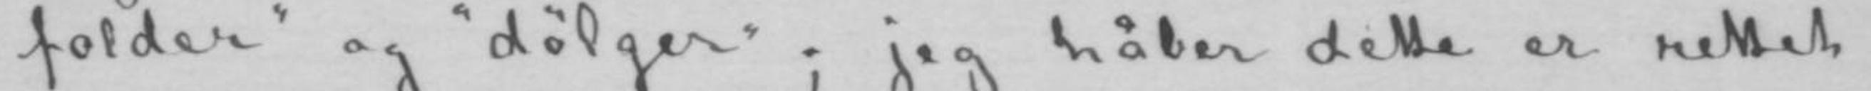«folder» og «dølger»; jeg håber dette er rettet;-
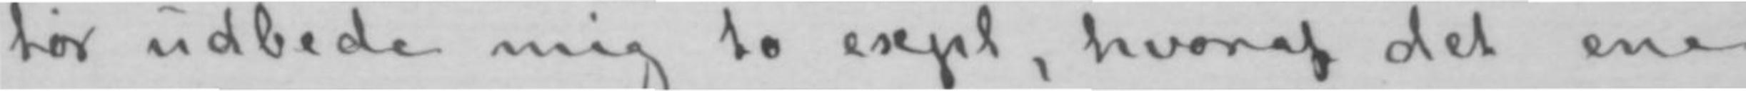tør udbede mig to expl, hvoraf det ene
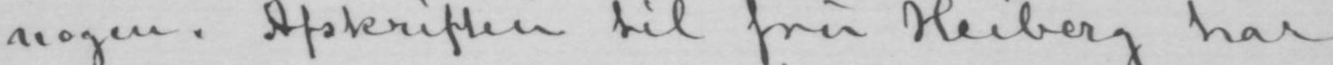nogen. Afskriften til fru Heiberg har
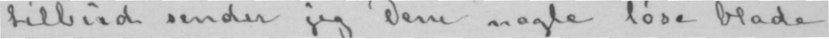tilbud sender jeg Dem nogle løse blade
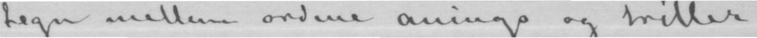tegn mellem ordene anings og triller,
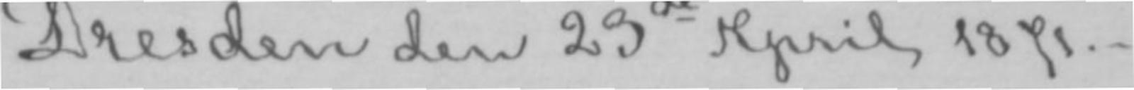Dresden den 23de April 1871.-
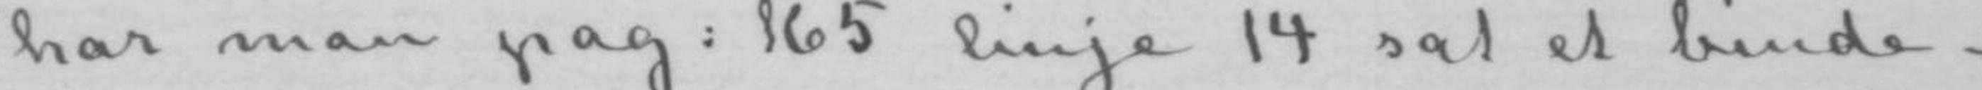har man pag: 165 linje 14 sat et binde-
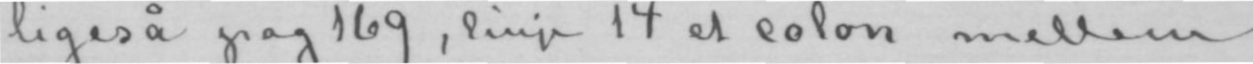ligeså pag 169, linje 14 et colon mellem
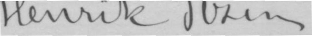Henrik Ibsen.
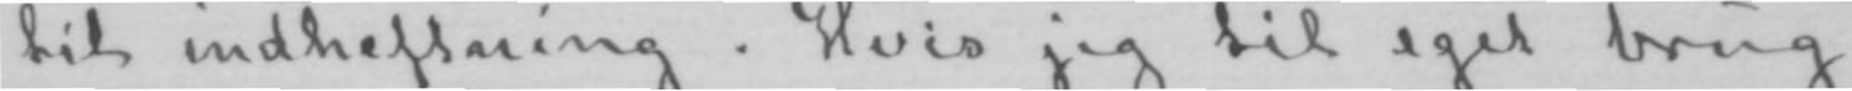til indheftning. Hvis jeg til eget brug
